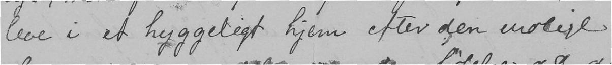leve i et hyggeligt hjem efter den urolige
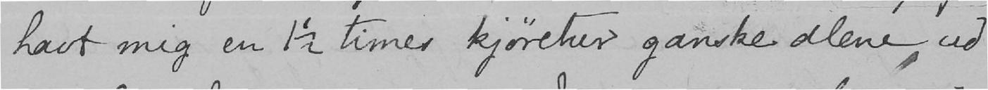havt mig en 1½ times kjøretur ganske alene ud
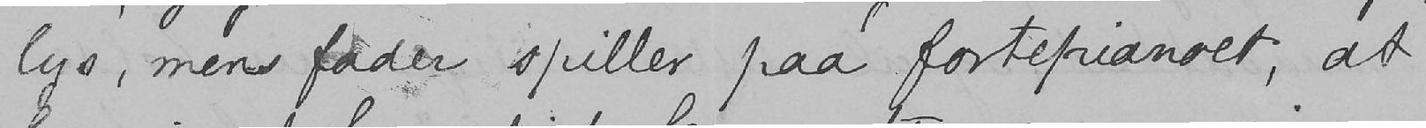lys, mens fader spiller paa fortepianoet, at
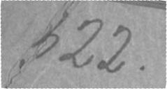322.
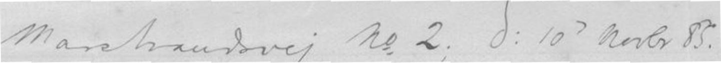Marstrandsvej no 2; :10 novbr. 85.
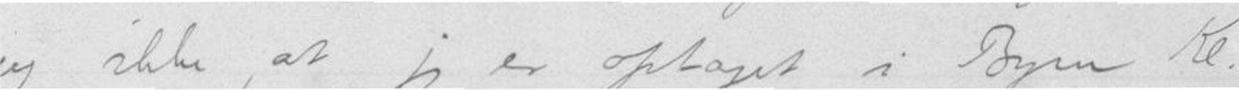jeg ikke, at jeg er optaget i Byen kl.
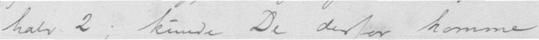halv 2; kunde De derfor komme
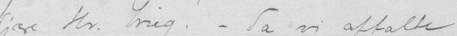Kjære Hr. Grieg! - Da vi aftalte
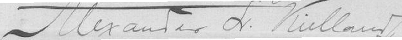Alexsander L. Kielland
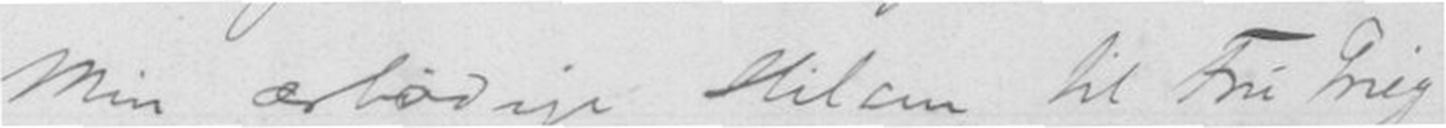Min ærbødige Hilsen til Fru Grieg;
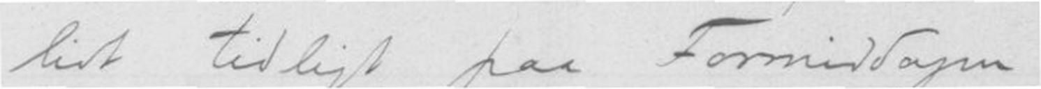lidt tidligt paa Formiddagen
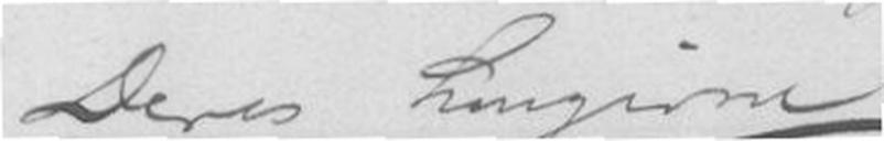Deres Hengivne
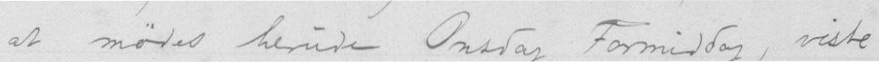at mødes herude Onsdag Formiddag, viste
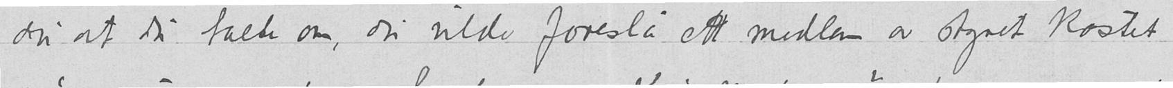du at du talte om, du vilde foreslå ett medlem av styret kastet
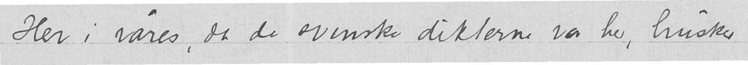Her i våres, da de svenske dikterne var her, husker
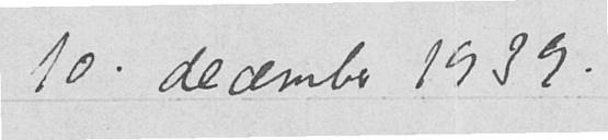10. december 1939.
